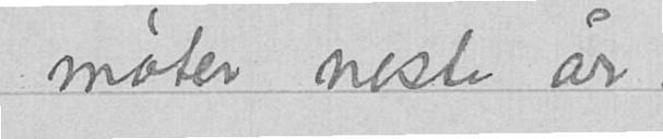møter neste år.
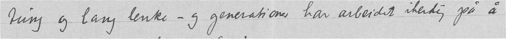tung og lang lenke – og generationer har arbeidet iherdig på å
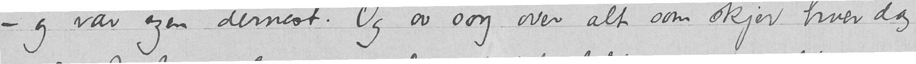– og vår egen dernest. Og av sorg over alt som skjer hver dag
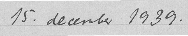15. december 1939.
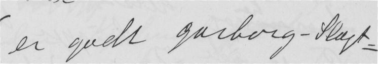er godt garborg— Slægt-
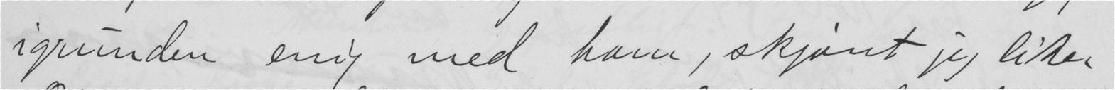igrunden enig med ham, skjønt jeg liker
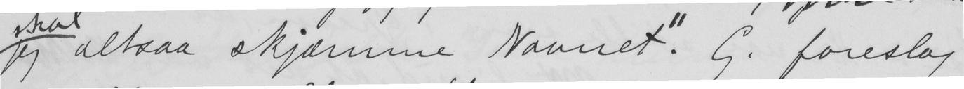jeg altsaa skjæmme Navnet". G. foreslog
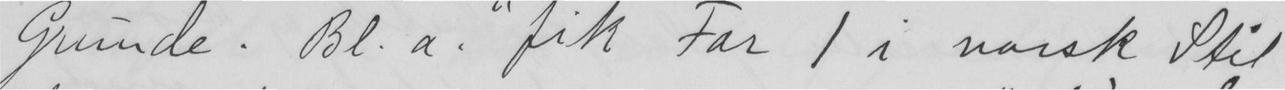Grunde. Bl. a. "fik Far 1 i norsk Stil
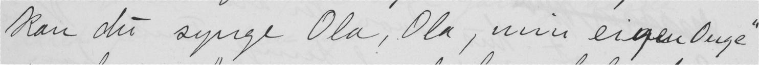kan du synge Ola, Ola, min eigen Onge"
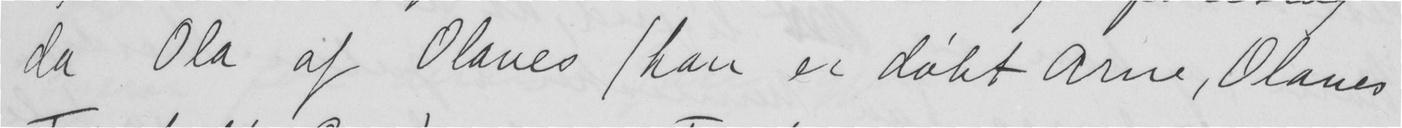da Ola af Olaves (han er døbt Arne, Olaves
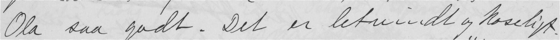Ola saa godt. Det er letvindt og koseligt.
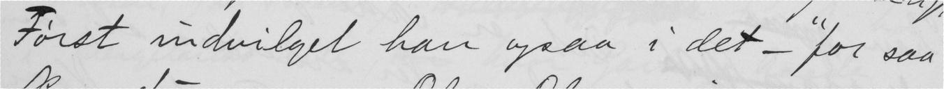Først indvilget han ogsaa i det - "for saa
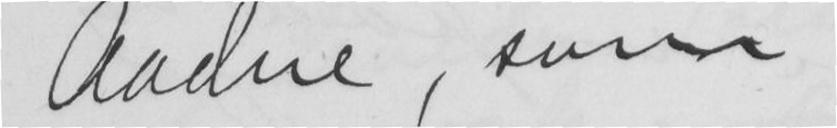Aadne, som
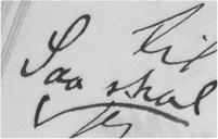Saa skal
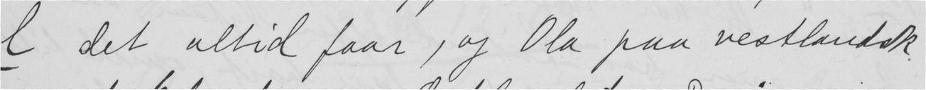l det altid faar, og Ola paa vestlandsk
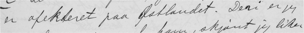er afekteret paa Østlandet. Deri er jeg
